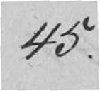45.
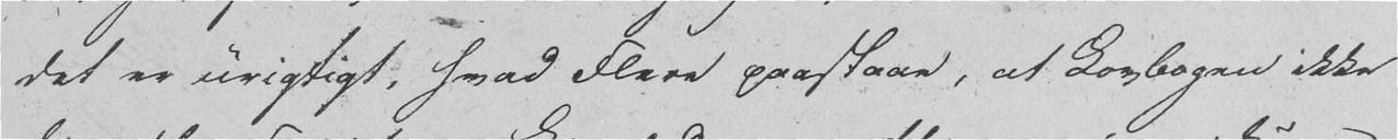det er urigtigt, hvad Flere paastaae, at Lovbogen ikke
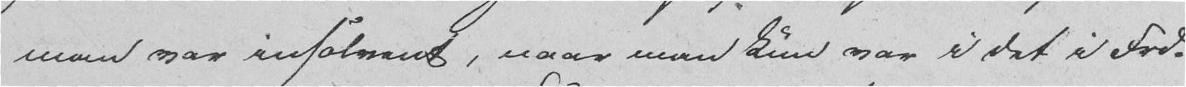man var insolvent, naar man kun var i det i Frd
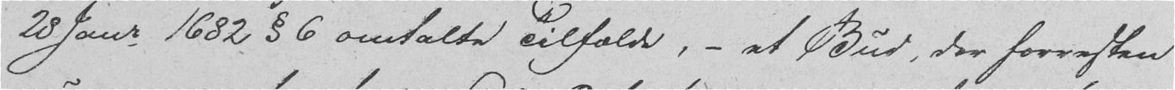28 Janr 1682 § 6 omtalte Tilfælde, – et Bud, der forresten
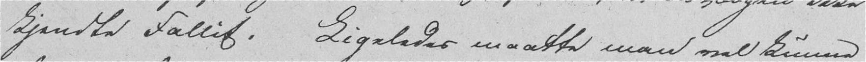kjendte Fallit. Ligeledes maatte man vel kunne
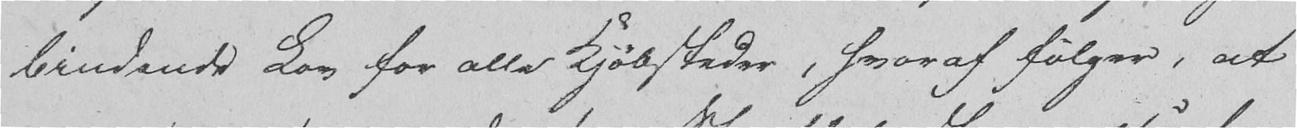bindende Lov for alle Kjøbsteder, hvoraf følger, at
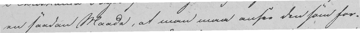en saadan Maade, at man maa ansee den som for-
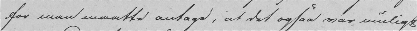for man maatte antage, at det ogsaa var muligt
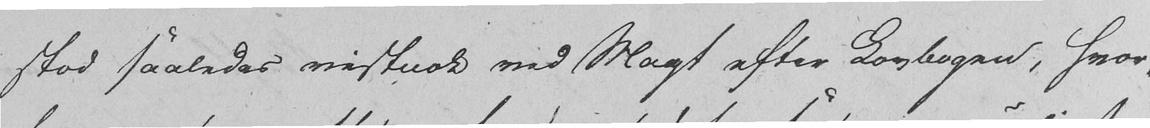stod saaledes vistnok ved Magt efter Lovbogen, hvor-
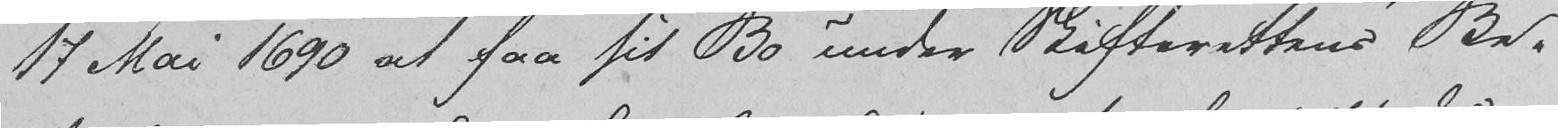17 Mai 1690 at faa sit Bo under Skifterettens Be-
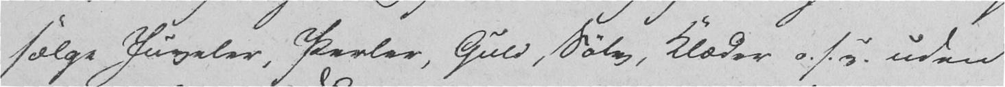sælge Juveler, Perler, Guld, Sølv, Klæder o. s. v. uden
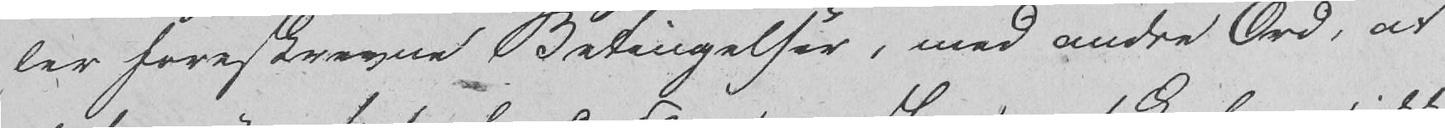ler foreskrevne Betingelser, med andre Ord, at
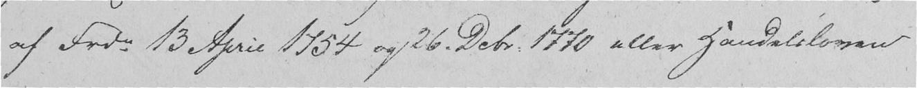af Frdn 13 April 1754 og 26. Dcbr. 1770 eller Handelsloven
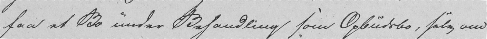faa et Bo under Behandling som Opbudsbo, selv om
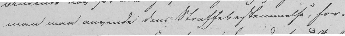man maa anvende dens Straffebestemmelse, for-
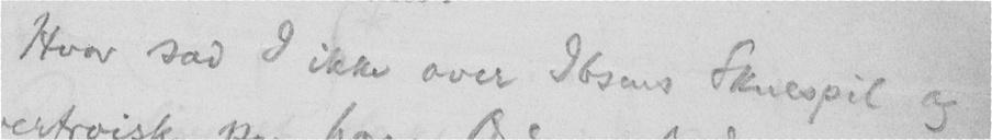Hvor sad I ikke over Ibsens Skuespil og
